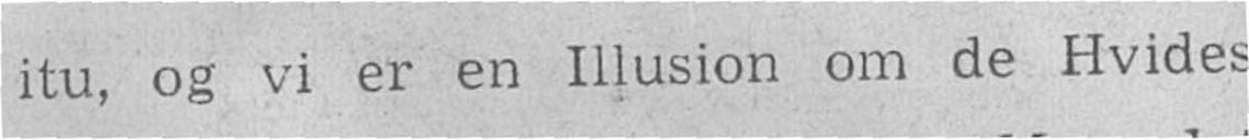itu, og vi er en Illusion om de Hvides
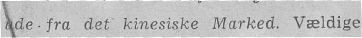Ade fra det kinesiske Marked. Vældige
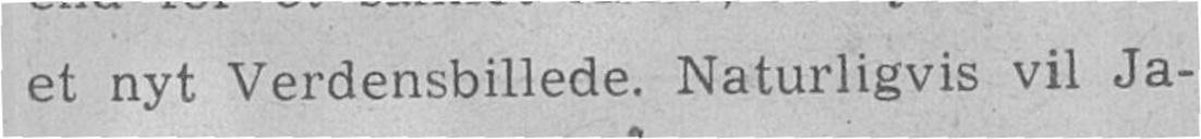et nyt Verdensbillede. Naturligvis vil Ja-
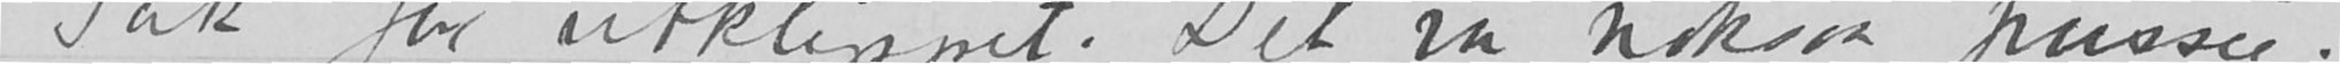Tak for utklippet. Det var noksaa pussig.
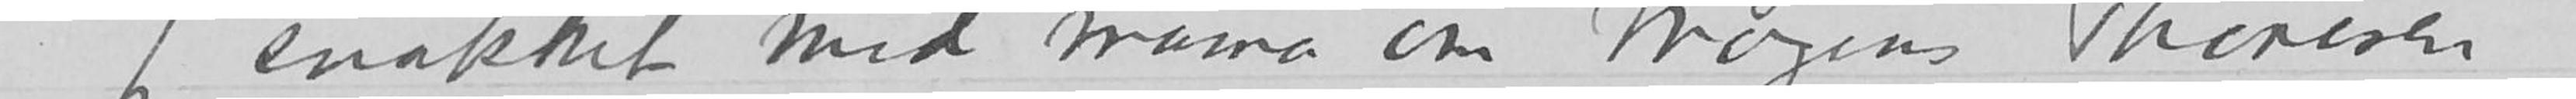Jeg snakket med mama om Mogens Thoresen
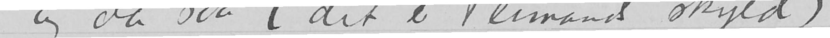og da saa (det er Peimands skyld).
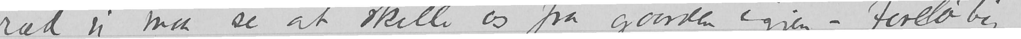ræd vi maa se at skille os fra gaarden igjen – foreløbig
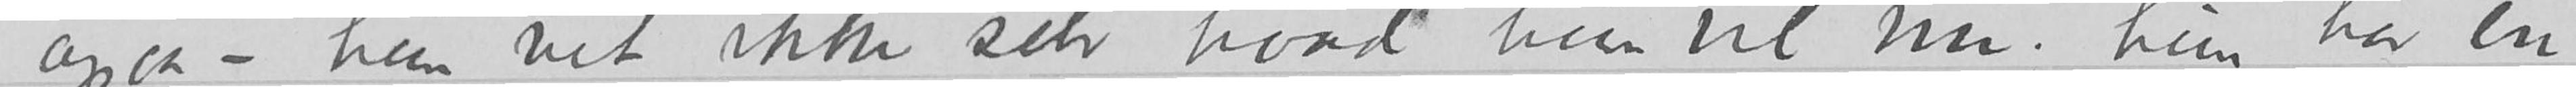ogsaa – hun vet ikke selv hvad hun vil nu, hun har en
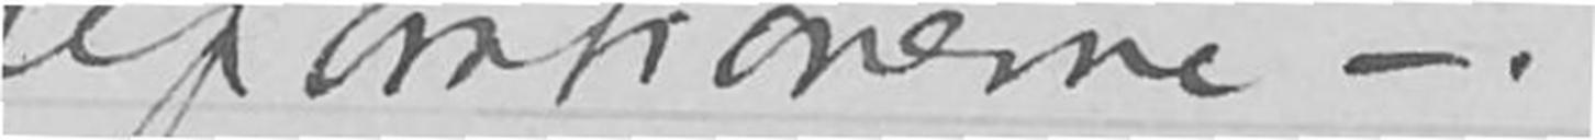reparationerne -.
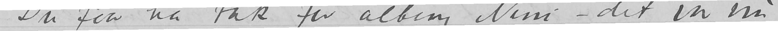Du faar ha tak for alting, Nini – det var nu
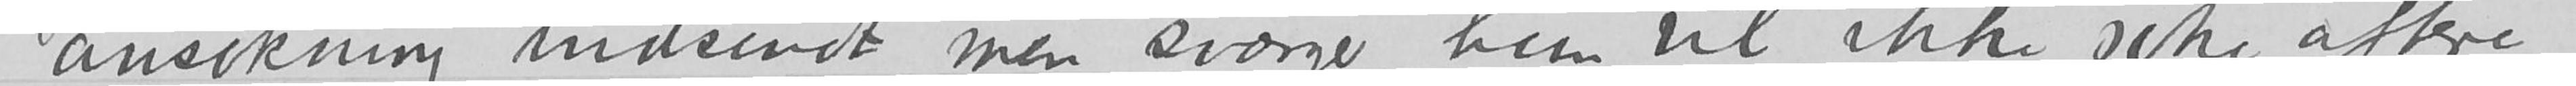ansøkning indsendt men sværger hun vil ikke søke oftere.
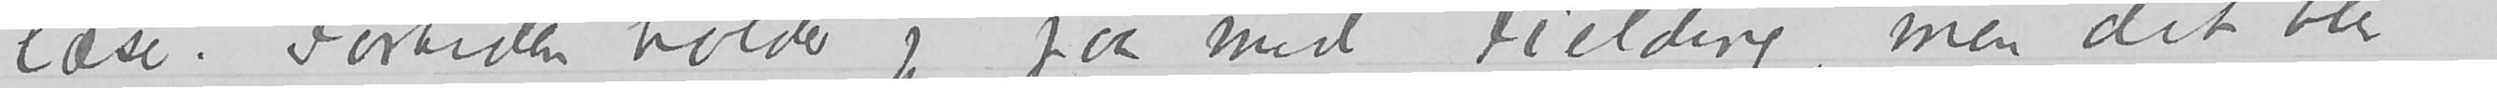læse. Fortiden holder jeg paa med Fielding, men det blir
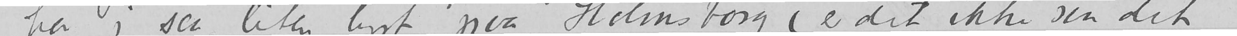har jeg saa liten lyst paa, Holmsborg (er det ikke saa det
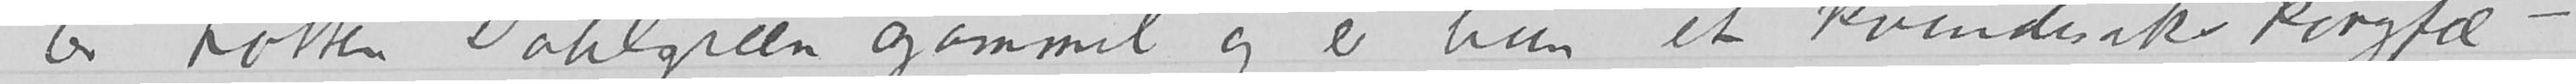Er Lotten Dahlgreen gammel og er hun et kvindesakskoryfæ - ?
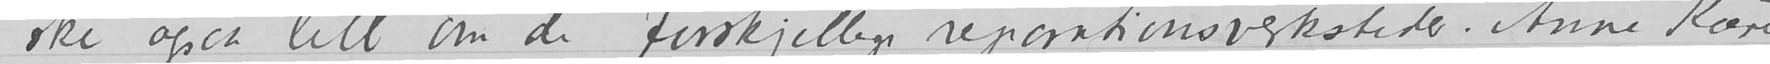ske ogsaa litt om de forskjellige reparationsverksteder. Anne Kure
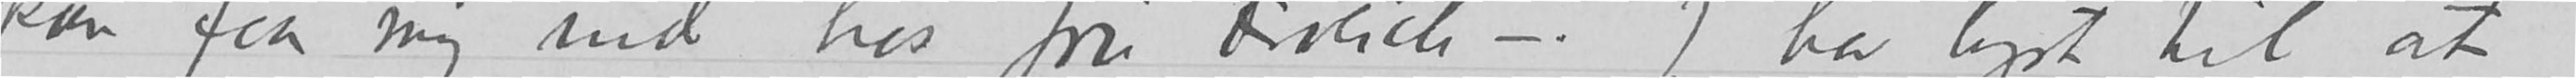kan faa mig ind hos fru Frølich -. Jeg har lyst til at
